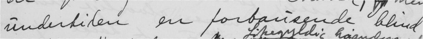undertiden en forbausende blind
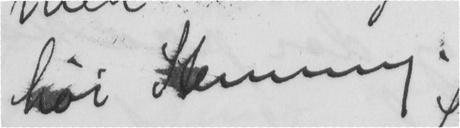høi Stemning.
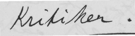Kritiker.
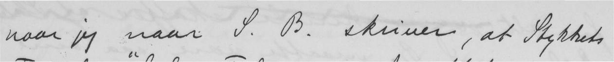naar jeg naar S. B. skriver, at Stykkets
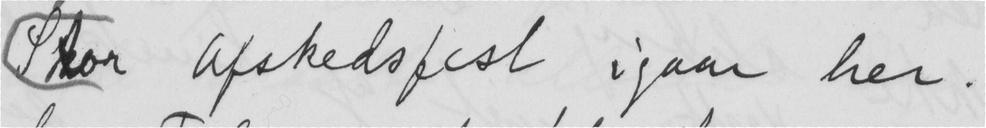Stor Afskedsfest igaar her.
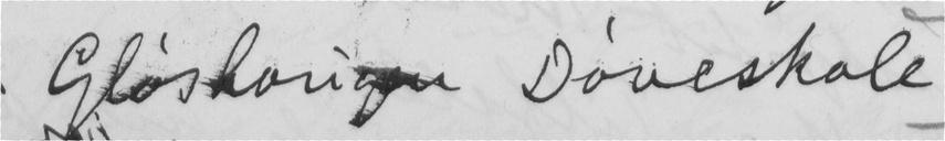Gløshaugen Doveskole
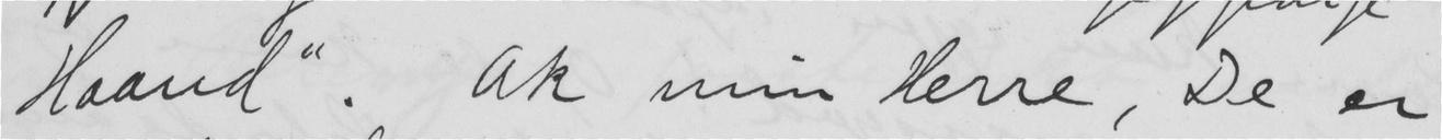Haand". Ak min Herre, De er
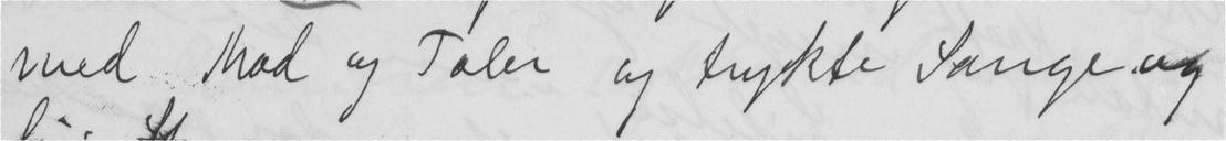med Mad og Taler og trykte Sange og
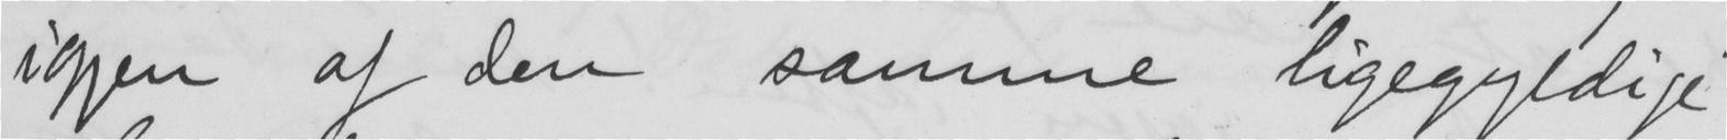igjen af den samme ligegyldige
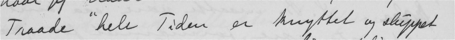Traade "hele Tiden er knyttet og sluppet
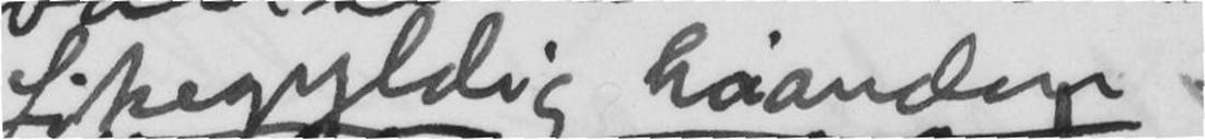Likegyldig haanden
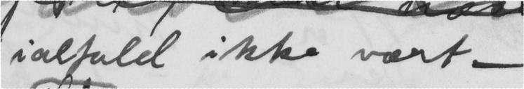ialfald ikke vært. -
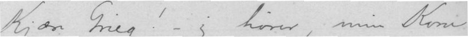Kjære Grieg! - jeg hører, min Kone
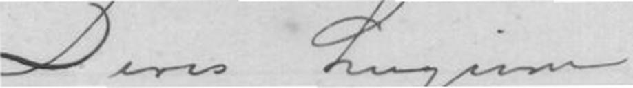Deres hengivne
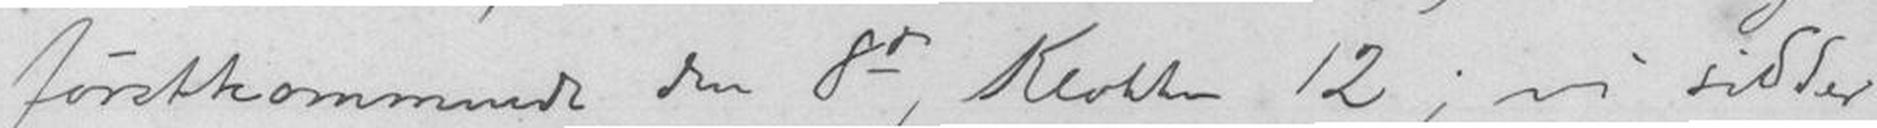førstkommende den 8de, Klokken 12; vi sidder
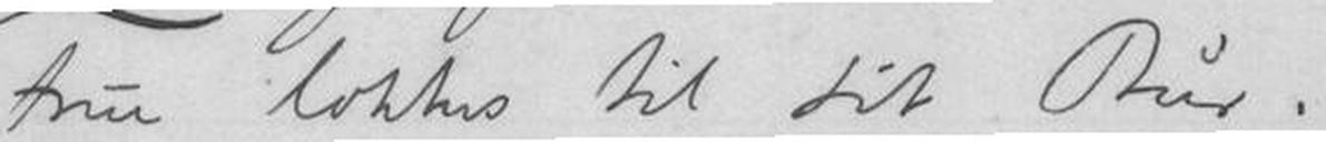Fru lokkes til dit
